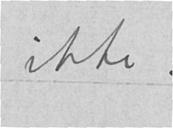ikke.
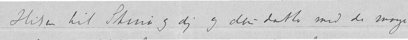Hilsen til Stina og dig og din datter med de mange
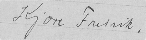Kjære Fredrik,
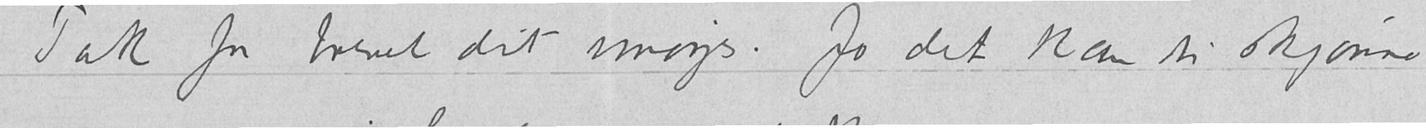Tak for brevet dit imorges. Jo det kan du skjønne
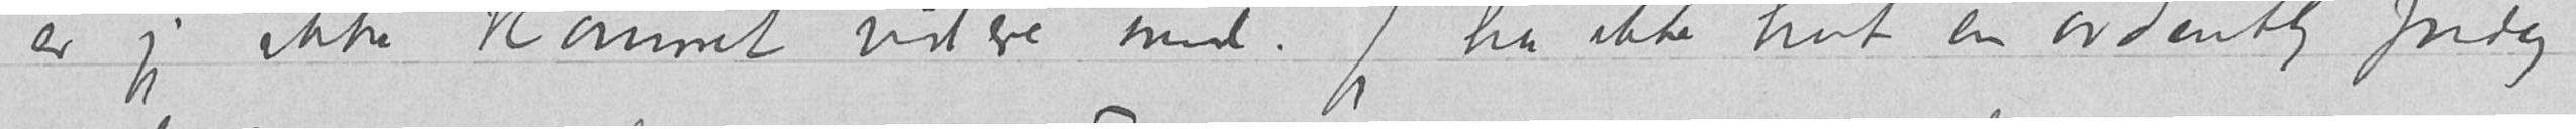er jeg ikke kommet videre med.  Jeg har ikke hat en ordentlig fridag
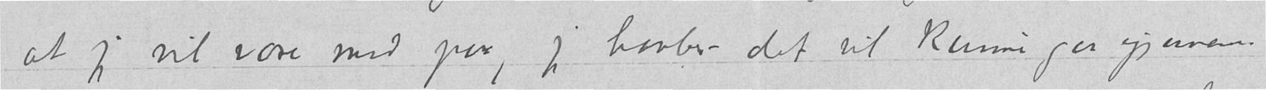at jeg vil være med paa, jeg haaber det vil kunne gaa igjennem.
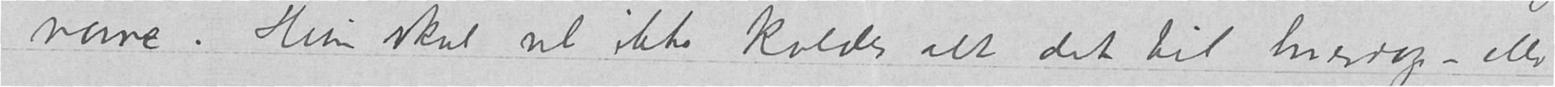navne. Hun skal vel ikke kaldes alt det til hverdags – eller
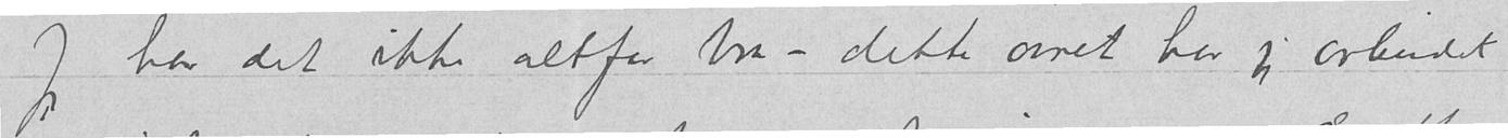Jeg har det ikke altfor bra - dette aaret har jeg arbeidet
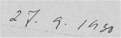27.9.1930
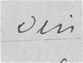Din
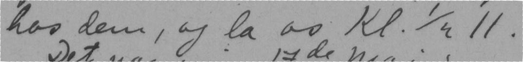hos dem, og la os Kl. 1/2 11.
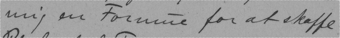mig en Formue for at skaffe
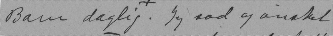Barn daglig. Jeg sad og ønsket
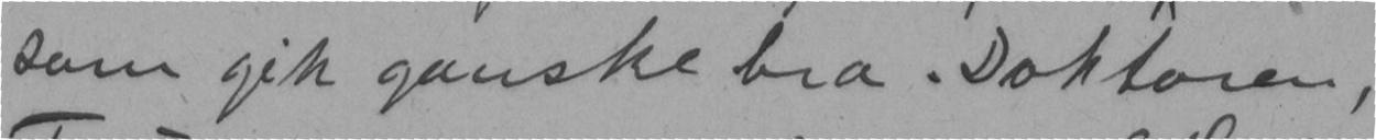som gik ganske bra - Doktoren,
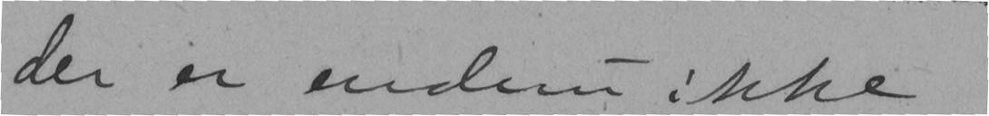der er endnu ikke
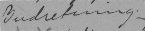Indretning.
